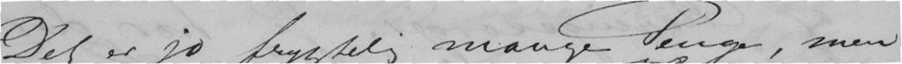Det er jo frygtelig mange Penge, men
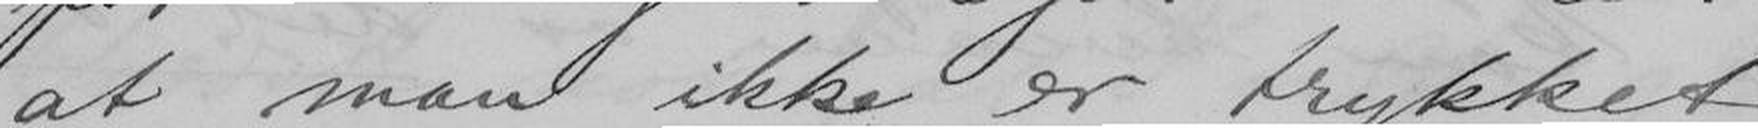at man ikke er trykket
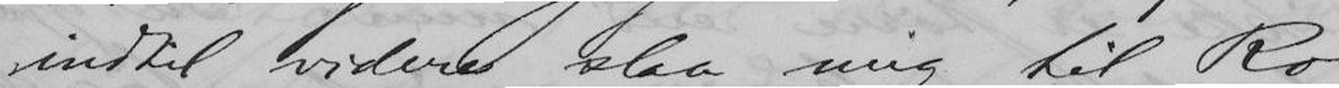indtil videre slaa mig til Ro
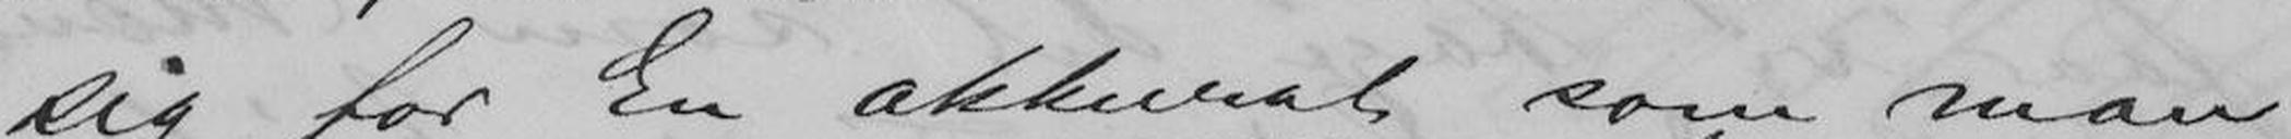sig for En akkurat som man
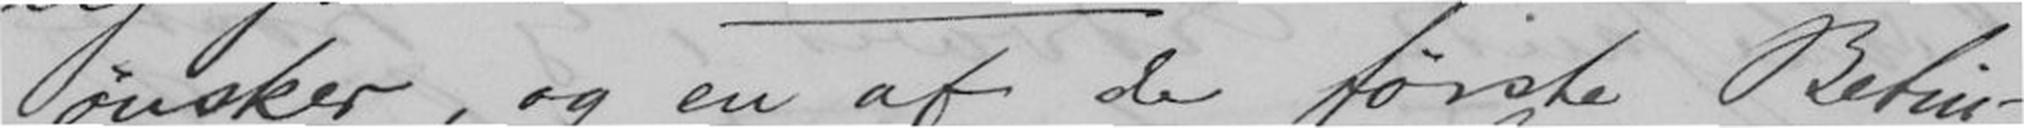ønsker, og en af de første Betin-
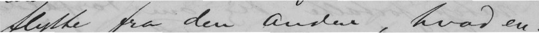flytte fra den Anden, hvad en-
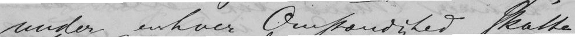under enhver Omstændighed skatte
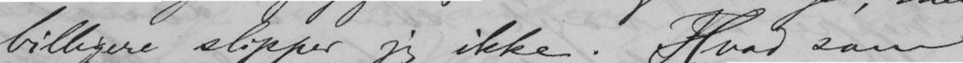billigere slipper jeg ikke. Hvad som
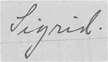Sigrid.
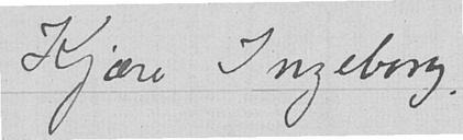Kjære Ingeborg,
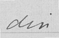din
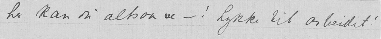her kan du altsaa se - ! Lykke til arbeidet!
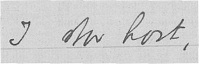I stor hast,
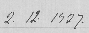2.12.1927.
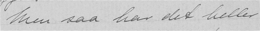Men saa har det heller
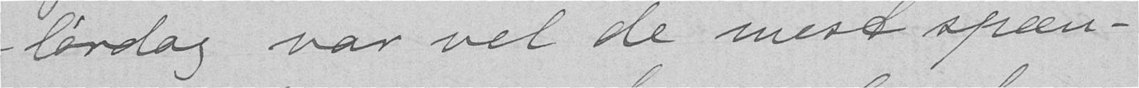- lørdag var vel de mest spæn-
